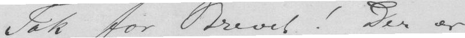Tak for Brevet! Der er
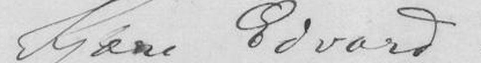Kjære Edvard!
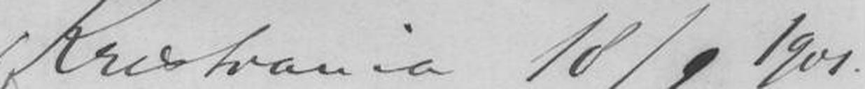Kristiania 18/9 1901.
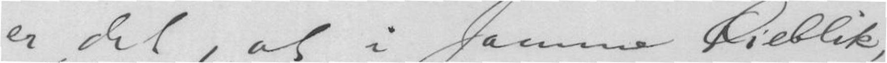er det, at i samme Øieblik,
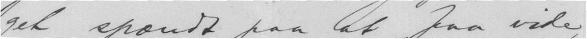get spændt paa at faa vide,
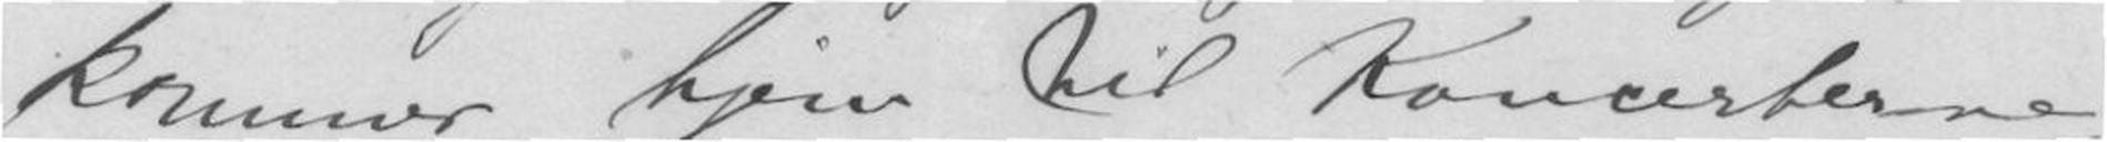kommer hjem til Koncerterne,
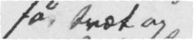så træt og
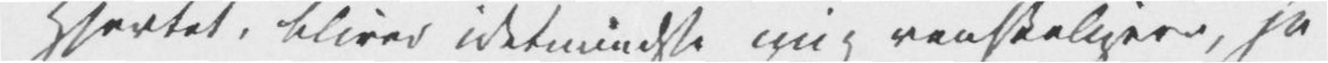Hjertet, bliver idetmindste mig vanskeligere, jo
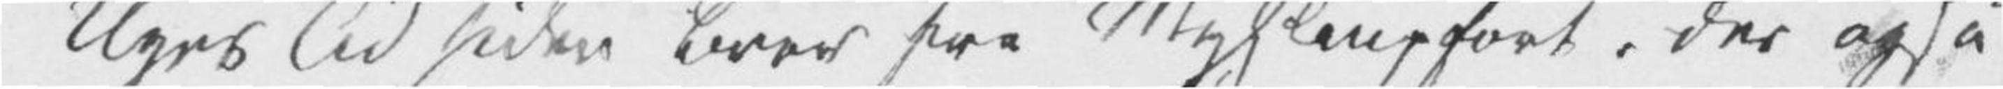Uges tid siden Brev fra Myhlenphort, der også
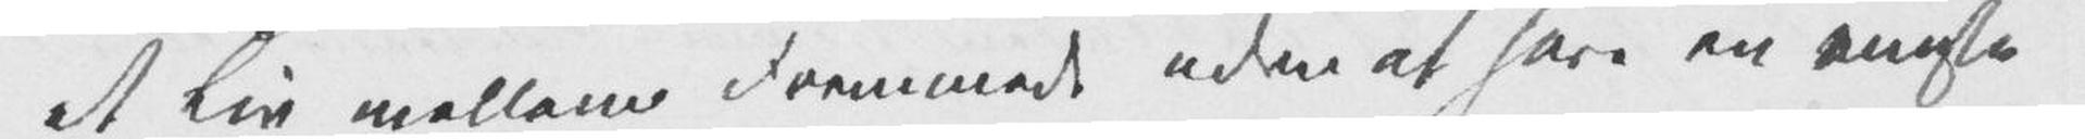et Liv mellem Fremmede uden at have en eneste
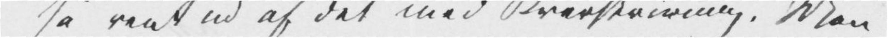så rent ud af det med Brevskrivning. Men
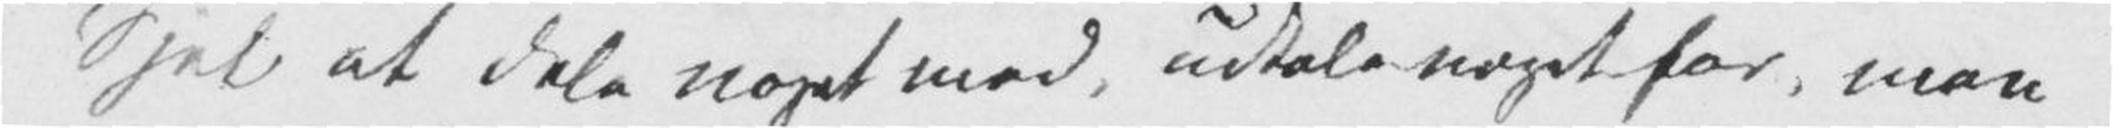Sjel at dele noget med, udtale noget for, man
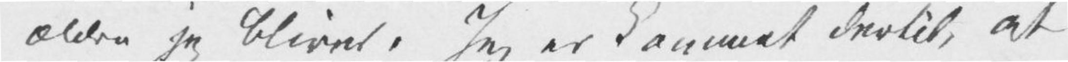ældre jeg bliver. Jeg er kommet dertil, at
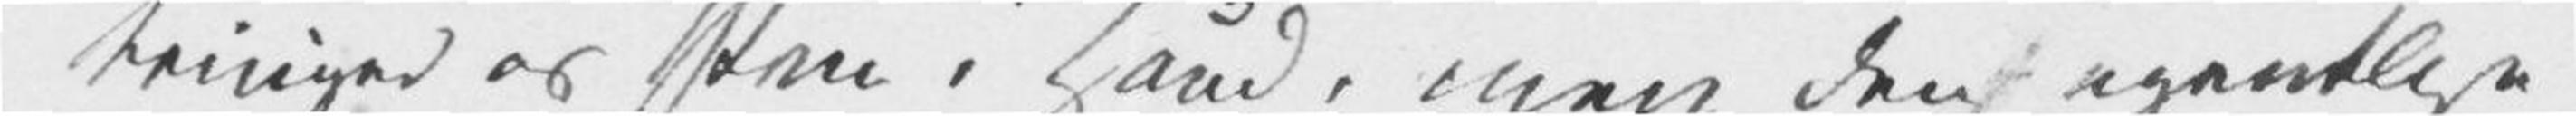tvinger os Pen i Hånd, men den egentlige
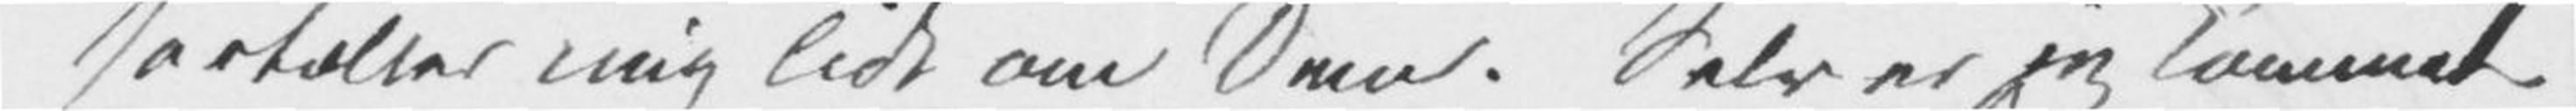fortæller mig lidt om Dem. Selv er jeg kommet
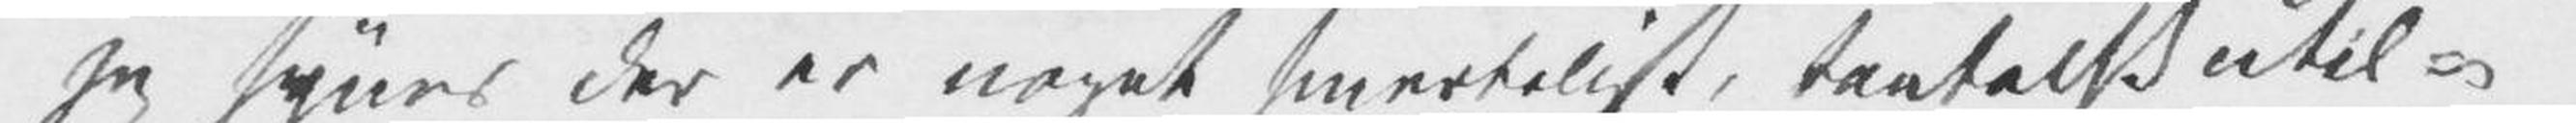jeg synes der er noget smerteligt, tantalsk util¬
